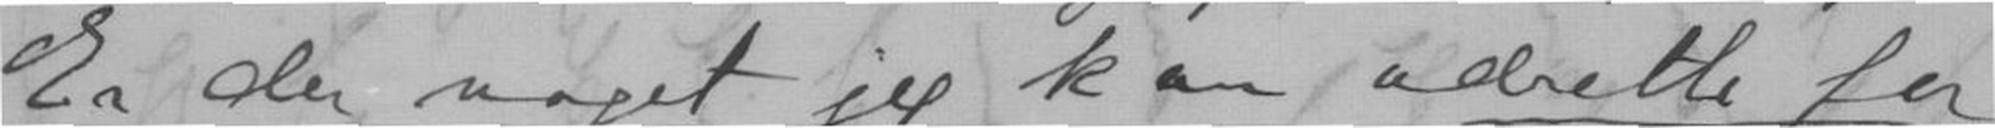Er der noget jeg kan udrette for
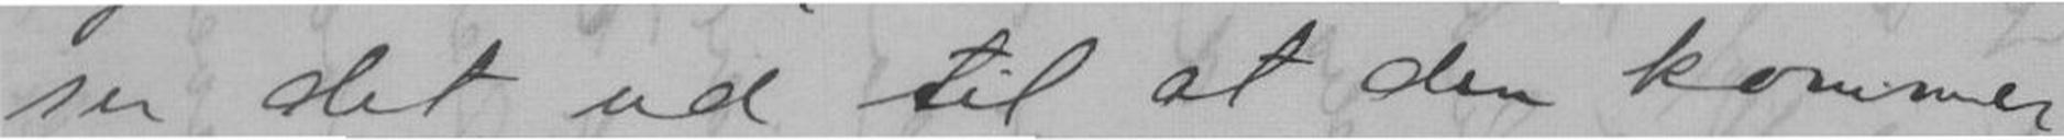ser det ud til at den kommer.
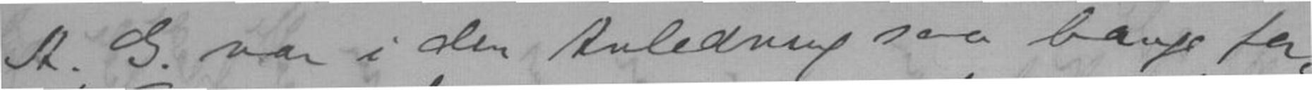A. G. var i den Anledning saa bange for,
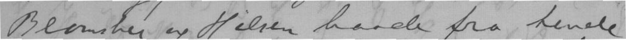Blomster og Hilsen baade fra hende
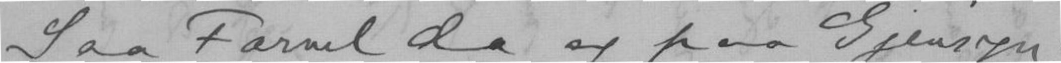Saa Farvel da og paa Gjensyn!
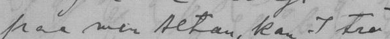Idag er her herligtpaa min Altan, kan I tro.
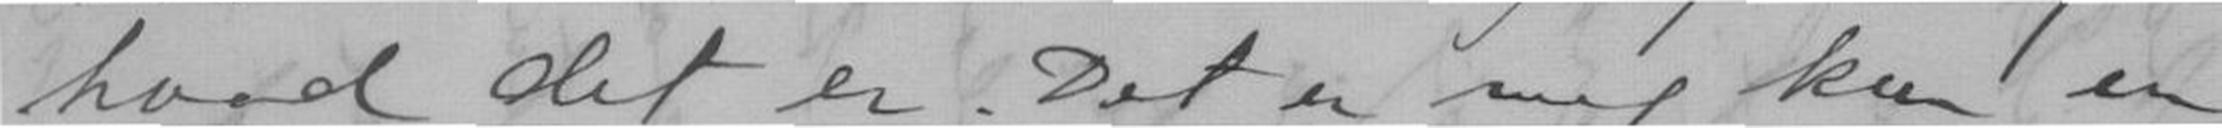hvad det er. Det er mig kun en
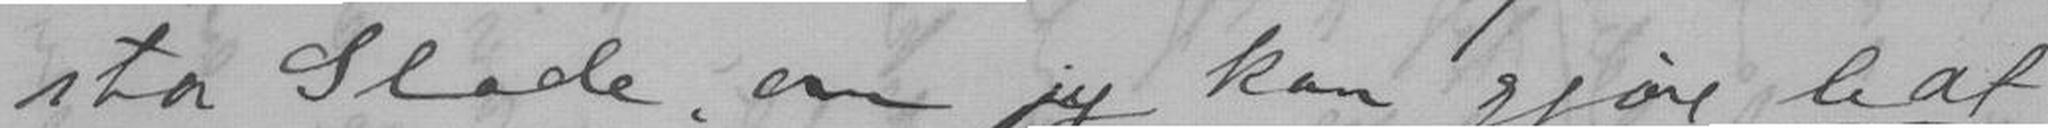stor Glæde, om jeg kan gjøre Lidt
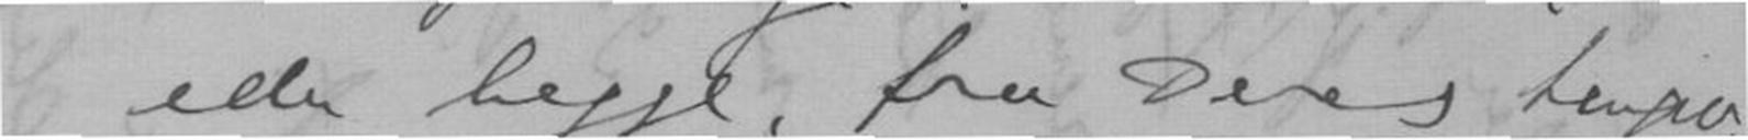eder begge, fra Deres hengiv.
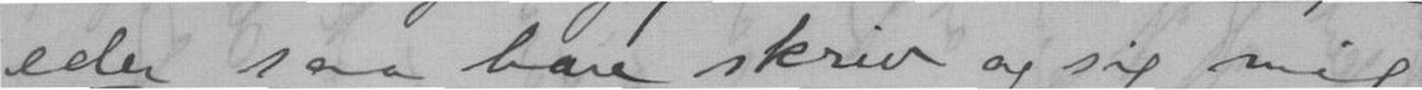eder saa bare skriv og sig mig
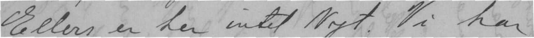Ellers er her intet Nyt. Vi har
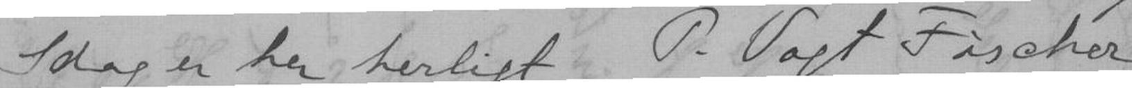P. Vogt Fischer
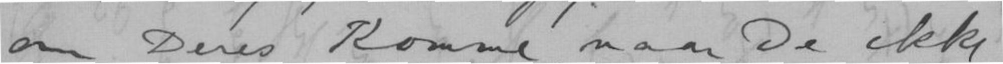om Deres Komme. naar De ikke
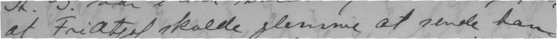at Fridtjof skulde glemme at sende ham
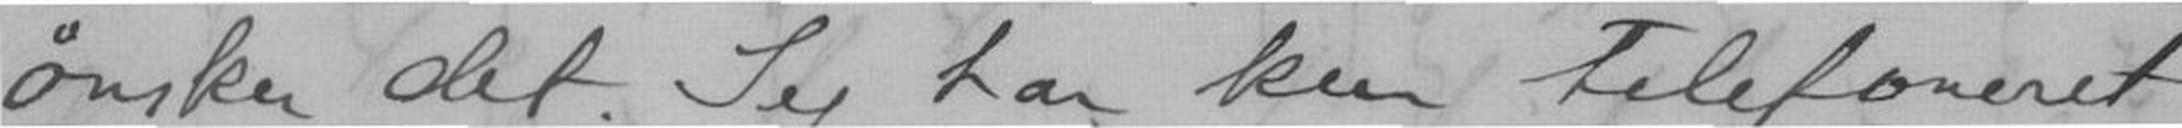ønsker det. Jeg har kun telefoneret
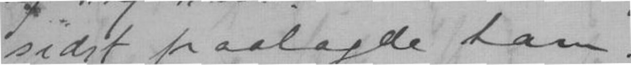sidst paalagde ham.
